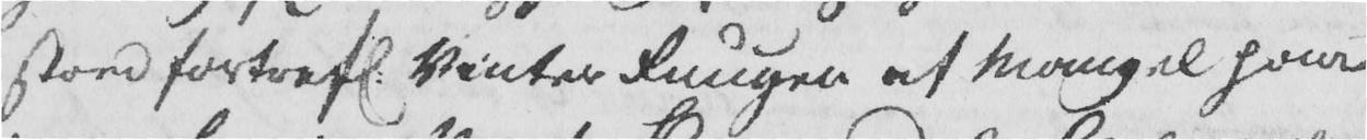stoed fortrefl. Vinter Ruugen af Mangel paa
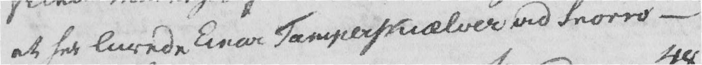at her levede Einar Tamperskiælver vide Snorro -
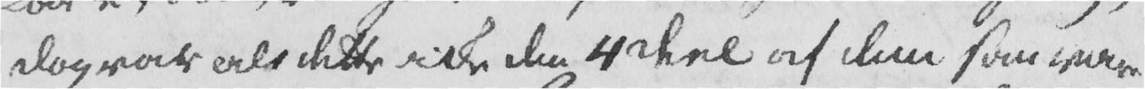dog var alt dette icke den 4 Del af dem som vare
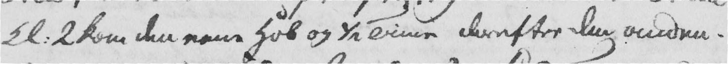kl. 2 kom den eene Hob og 1/2 Time derefter den anden.
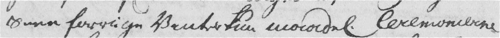Snee forrige Vinter kun maadel. Ceremonierne
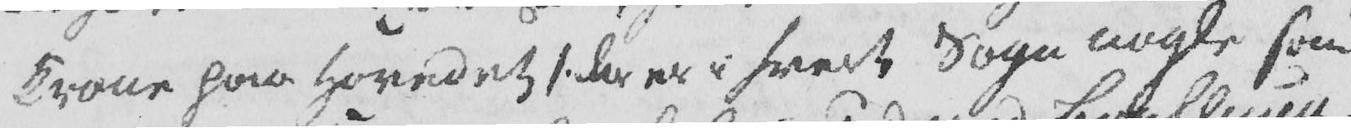Krone paa Hovedet /: der er i hvert Sogn nogle som
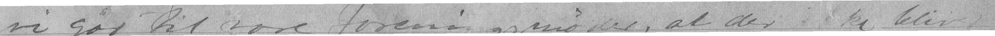vi går til vore foreninsmøder, at der ikke bliver
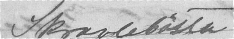Skravlebøssa
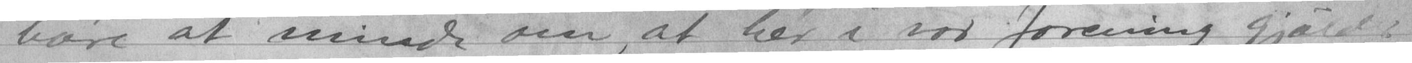bare at minde om, at her i vor forening gjelds
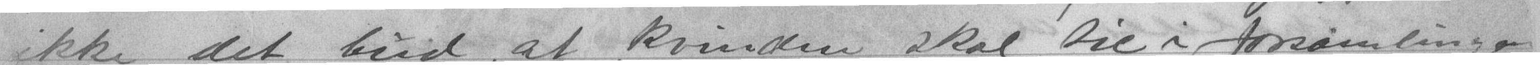ikke det bud, at "kvinden skal tie i forsamlinger
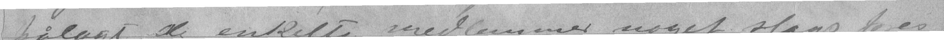pålagt de enkelte medlemmer noget slags fores
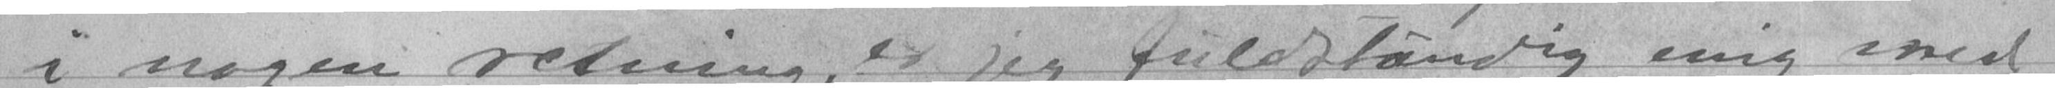i nogen retning, er jeg fulstendig enig med
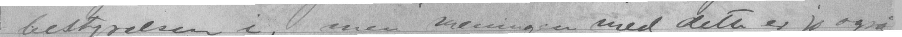bestyrelsen i, men meningen med dette er jo også
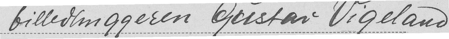billedhuggeren Gustav Vigeland
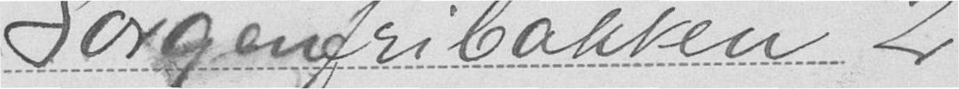Sorgenfribakken 2
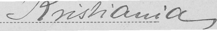Kristiania
